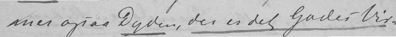mer ogsaa Dyden, der er det Godes Vir-
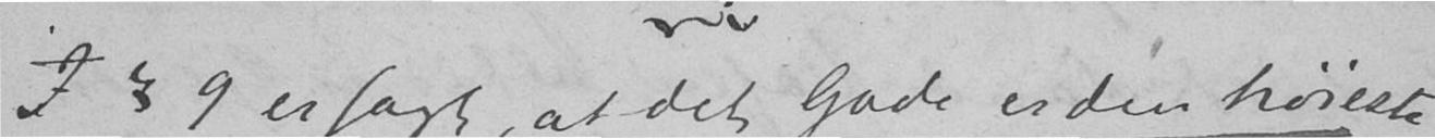I § 9 er sagt, at det Gode er den høieste
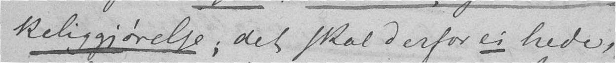keliggjørelse; det skal derfor ei hede,
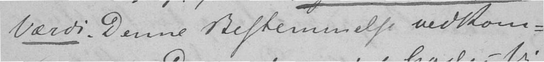Værdi. Denne Bestemmelse vedkom-
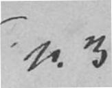p. 3
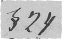§ 24.
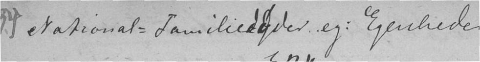National-Familiedyder eg: Eenheder.
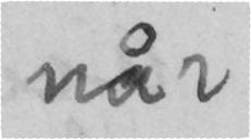når
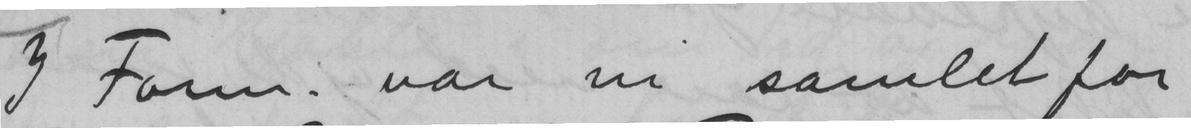I Form. var vi samlet for
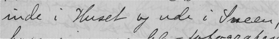inde i Huset og ude i Sveen,
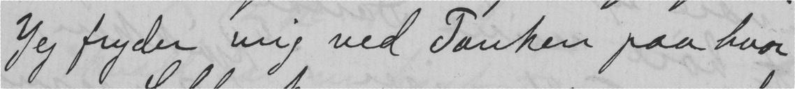Jeg fryder mig ved Tanken paa hvor
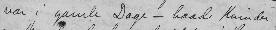var i gamle Dage - baade Kvinder
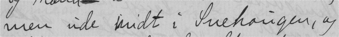men ude midt i Snehaugen, og
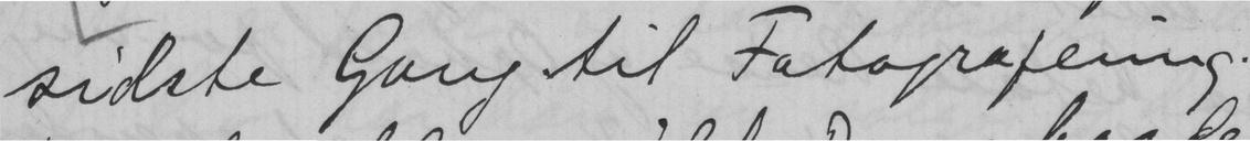sidste Gang til Fotografering.
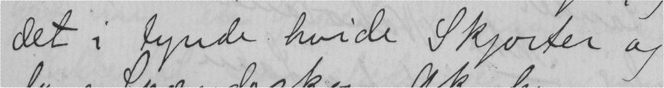det i tynde hvide Skjorter og
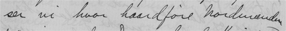ser vi hvor haardføre Nordmændene
